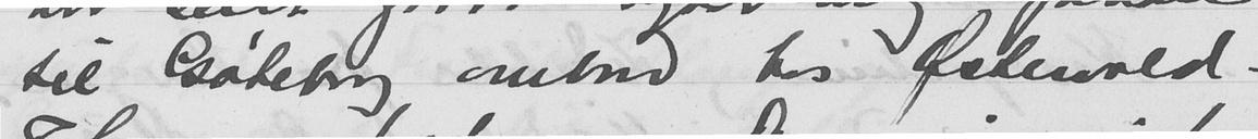til Gøteborg ombord hos Østervold.
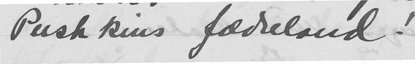Pushkins fædreland!
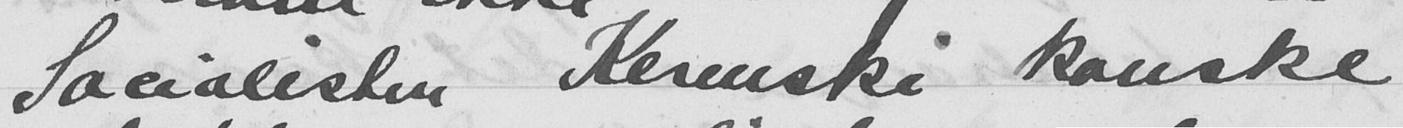Socialisten Kerenski kanske
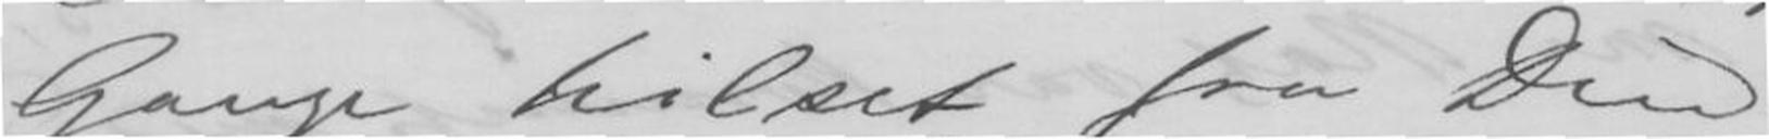Gange tilset fra Din
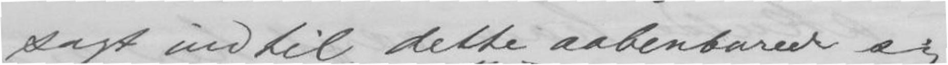sagt indtil dette aabenbaret sig
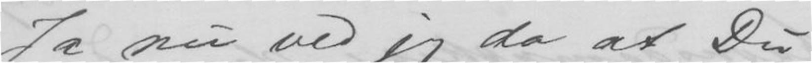Ja nu ved jeg da at Du
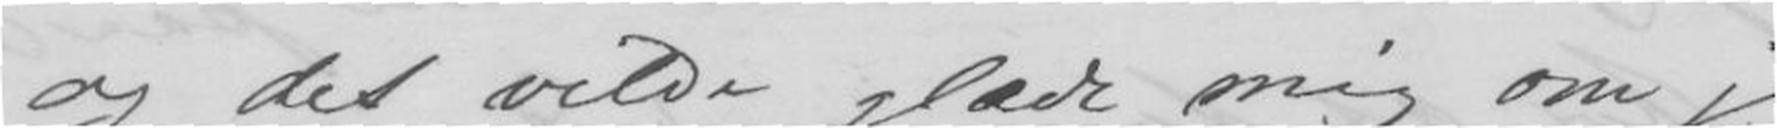og det vilde glæde mig om jeg
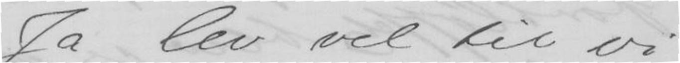Ja lev vel til vi
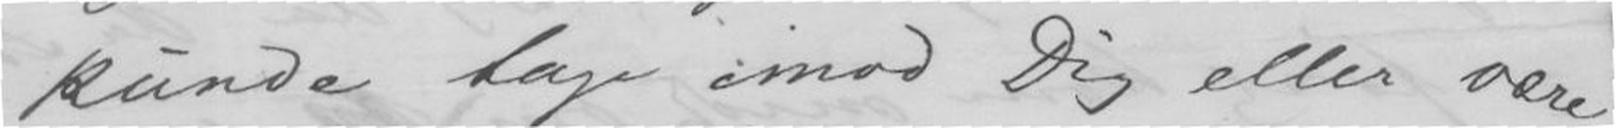kunde tage imod Dig eller være
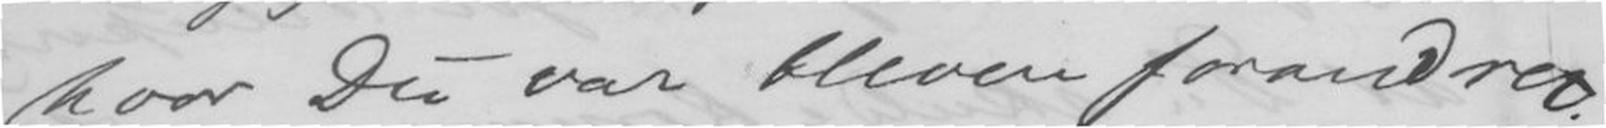hvor Du var bleven forandret.
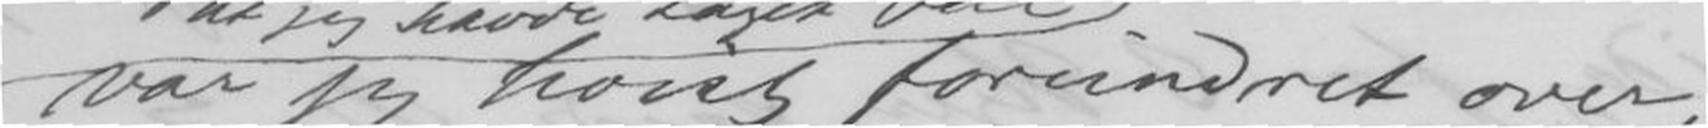var jeg høist forundret over,
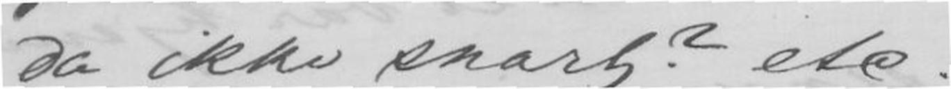da ikke snart? etc.
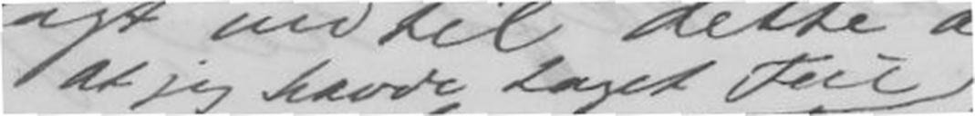at jeg havde taget Feil
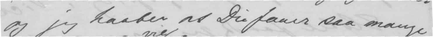og jeg Haaber at Du faaer saa mange
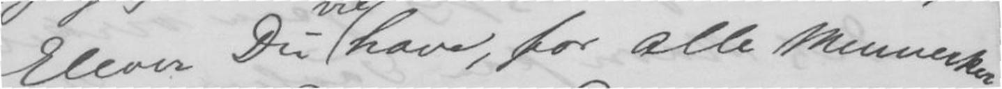Elever Du have, for alle Mennesker
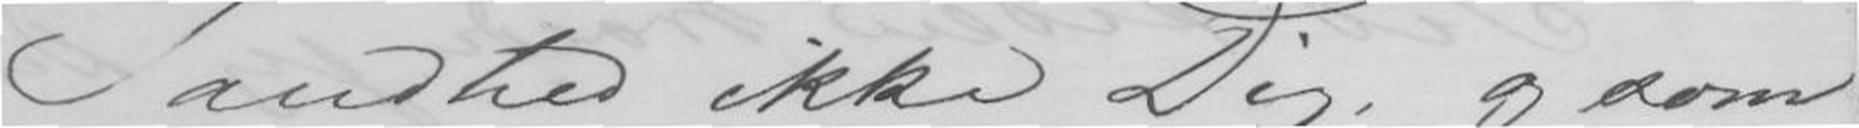Sandhed ikke Dig, og som
